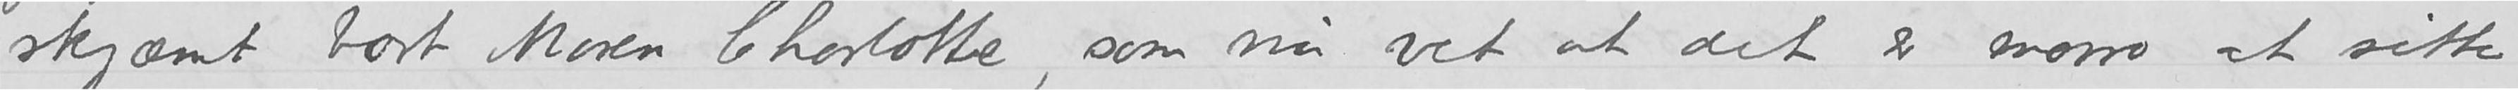skjæmt bort Maren Charlotte, som nu vet at det er moro at sitte
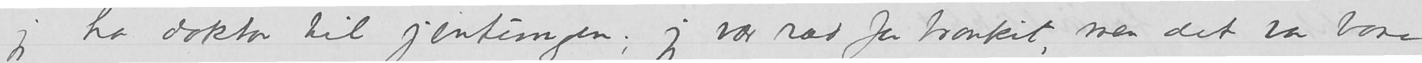jeg ha doktor til jentungen; jeg var ræd for bronkit, men det var bare
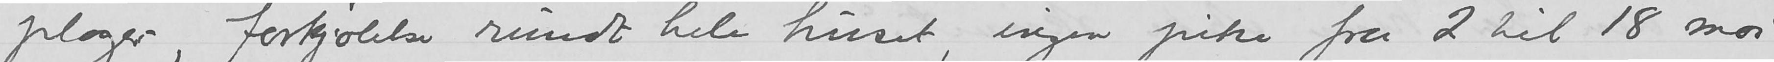plager, forkjølelse rundt hele huset, ingen pike fra 2 til 18 mai,
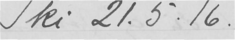Ski 21.5.16
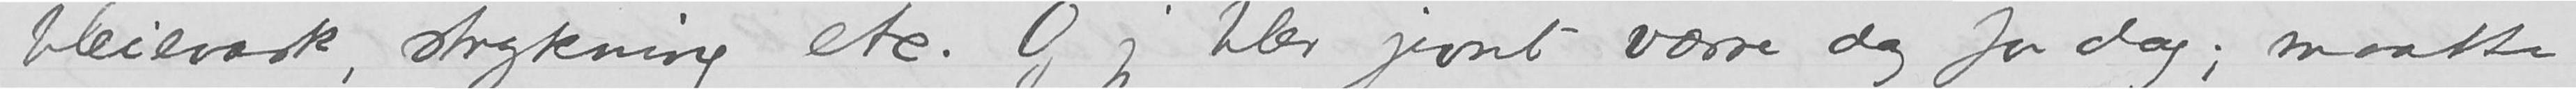bleievask, strykning etc. Og jeg blev jevnt værre dag for dag; maatte
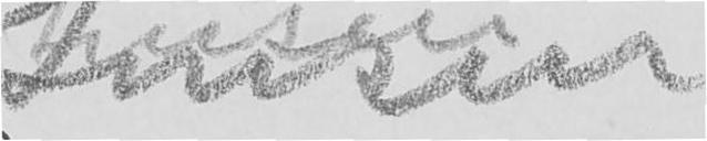Sinsen
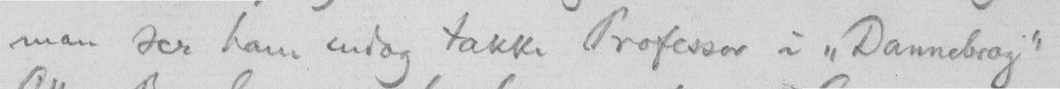man ser ham endog takke Professor i "Dannebrog"
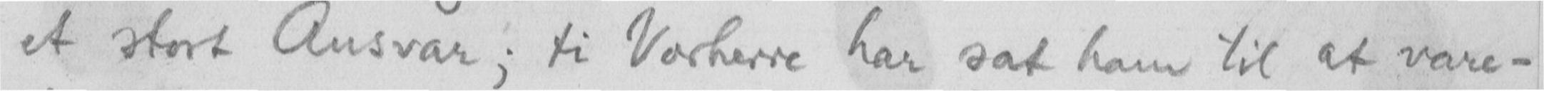et stort Ansvar; ti Vorherre har sat ham til at vare-
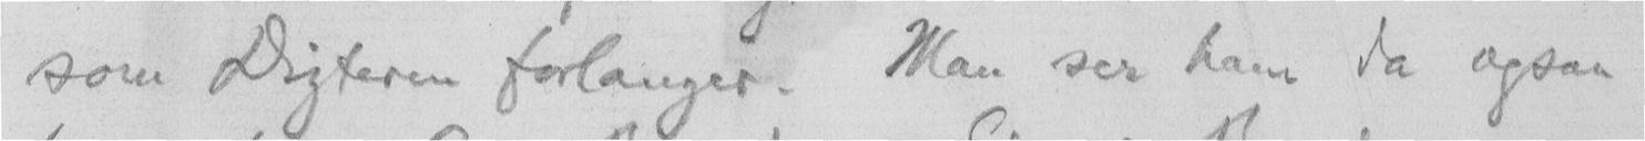som Digteren forlanger. Man ser ham da ogsaa
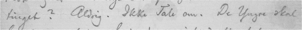tinget? Aldrig. Ikke Tale om. De Yngre skal
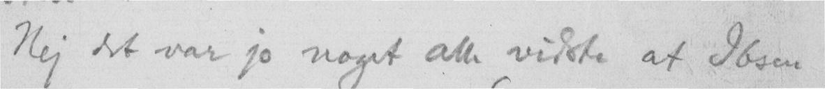Nej det var jo noget alle vidste at Ibsen
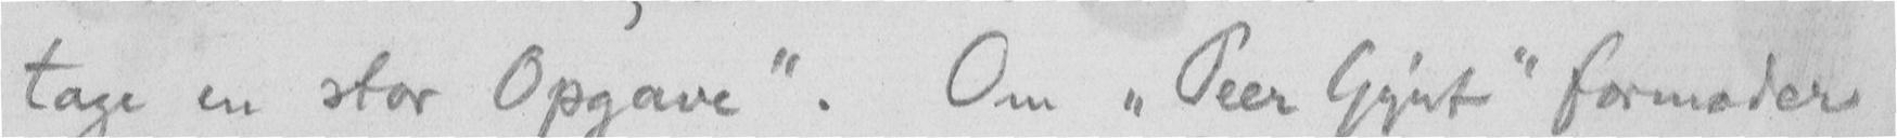tage en stor Opgave". Om "Peer Gynt" formoder
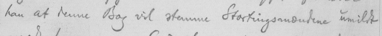han at denne Bog vil stemme Stortingsmændene umildt
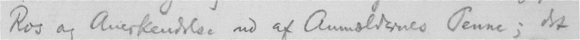Ros og Anerkendelse ud af Anmeldernes Penne; det
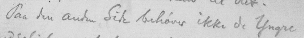Paa den anden Side behøver ikke de Yngre
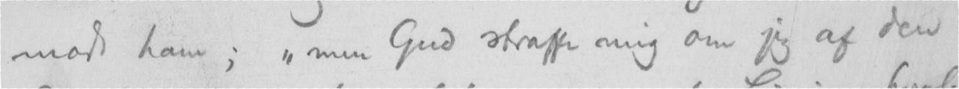mod ham; "men Gud straffe mig om jeg af den
